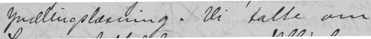yndlingslæsning. Vi talte om
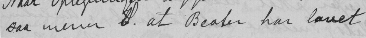saa mener S. at Beater har lavet
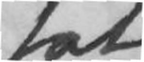fat
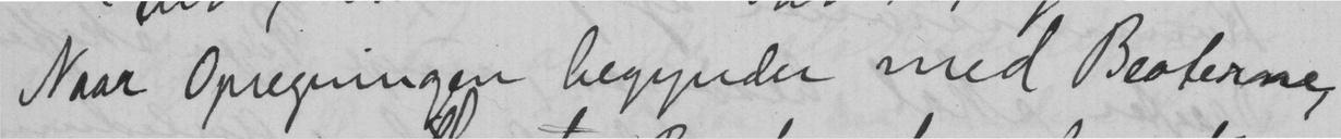Naar Opregningen begynder med Beaterne,
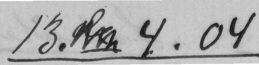13. 4.04
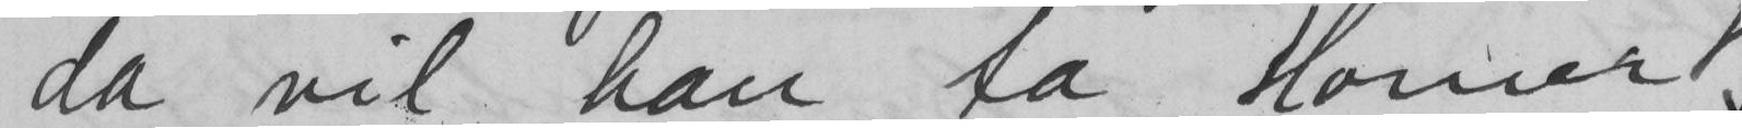da vil han ta Homer
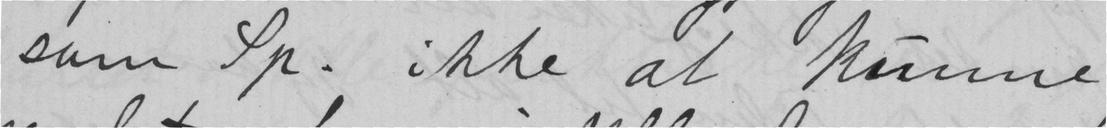som Sp. ikke at kunne
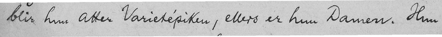blir hun atter Varietépiken, ellers er hun Damen. Hun
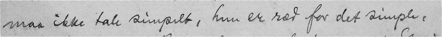maa ikke tale simpelt, hun er ræd for det simple,
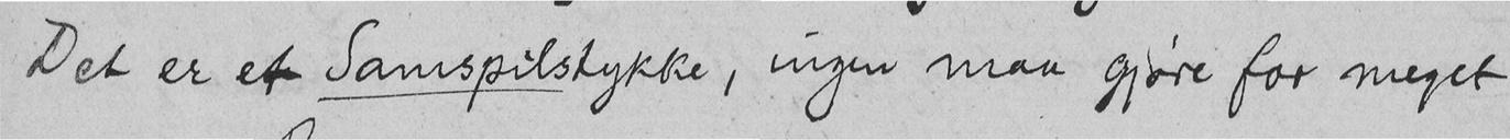Det er et Samspilstykke, ingen maa gjøre for meget
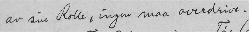av sin Rolle, ingen maa overdrive.
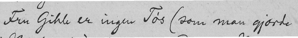Fru Gihle er ingen Tøs (som man gjorde
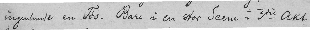ingenlunde en Tøs. Bare i en stor Scene i 3die Akt
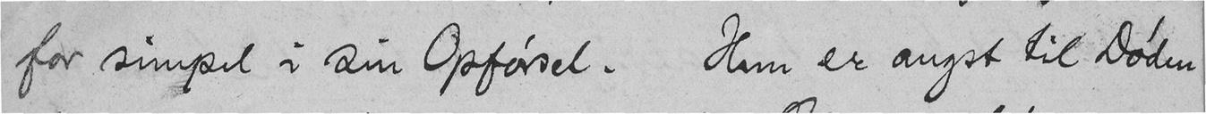for simpel i sin Opførsel. Hun er angst til Døden
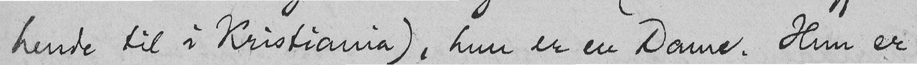hende til i Kristiania), hun er en Dame. Hun er
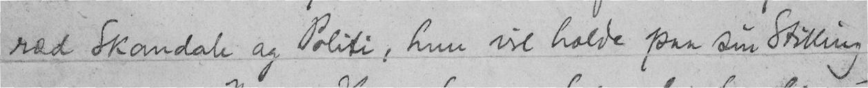ræd Skandale og Politi, hun vil holde paa sin Stilling
